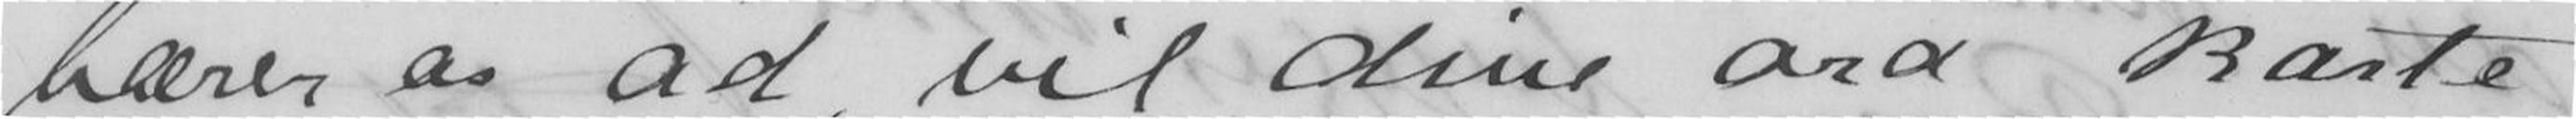bærer os ad, vil dine ord kaste
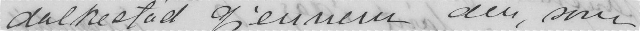dolkestød gjennem den, som
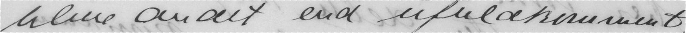blive amdet end ufuldkomment.
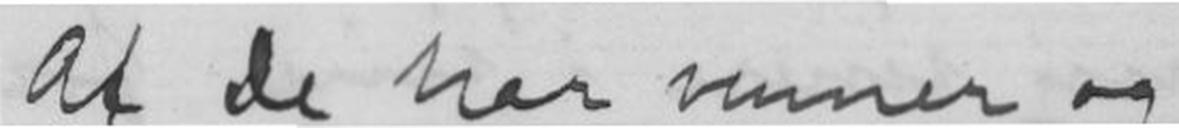At De har venner og
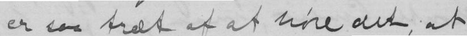er saa træt af at høre det, at
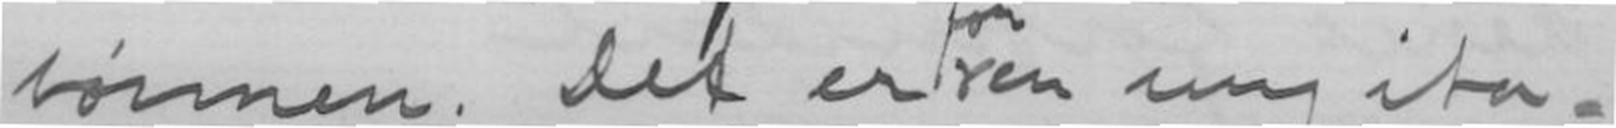bønnen. Det er for en ung ita-
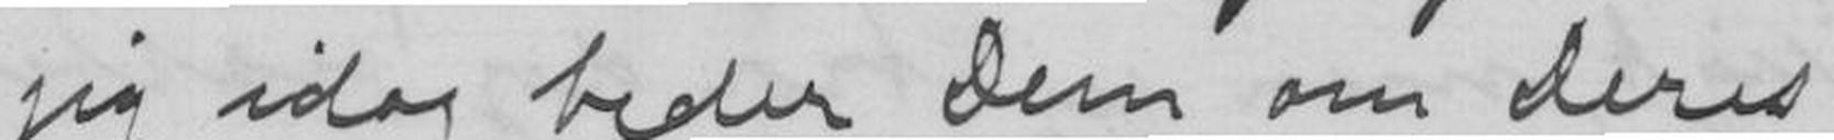jeg idag beder Dem om Deres
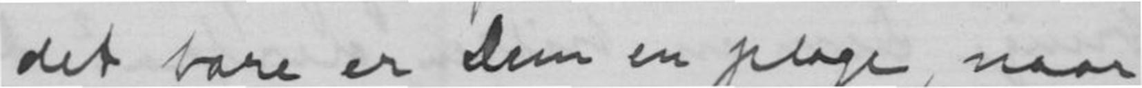det bare er Dem en plage, naar
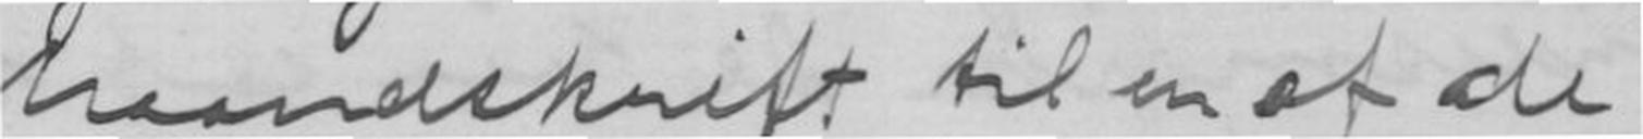haandskrift til en af de
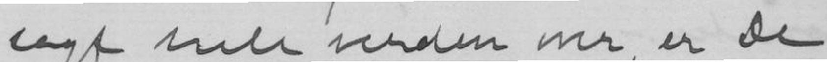sagt hele verden over, er De
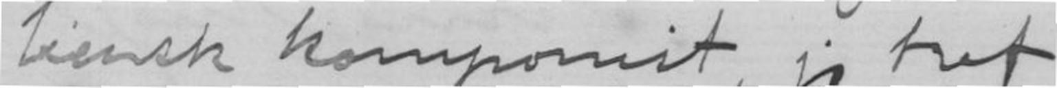liensk komponist, jeg traf
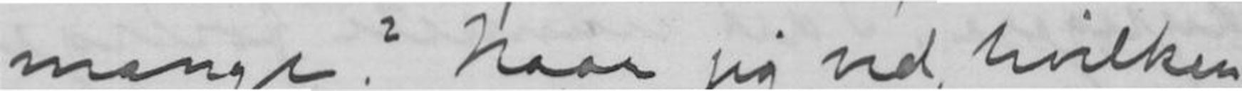mange? Naar jeg ved, hvilken
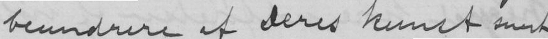beundrere af Deres kunst rent
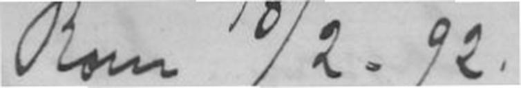Rom 18/2-92.
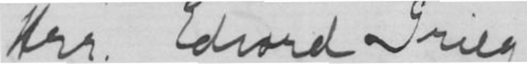Hrr. Edvard Grieg!
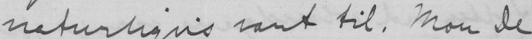naturligvis vant til. Mon De
